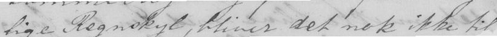lige Regnskyl, bliver det nok ikke til
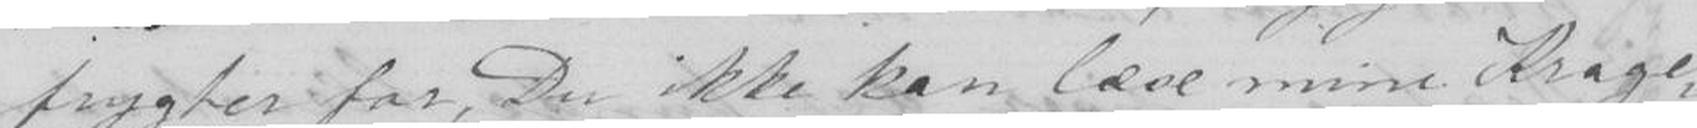frygter for, Du ikke kan læse mine. Krage,
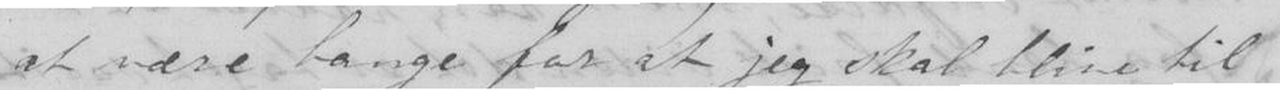at være bange for at jeg skal blive til
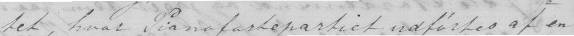tet, hvor Pianofortepartiet udføres af en
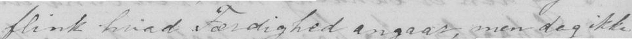flink hvad Færdighed angaar, men dog ikke
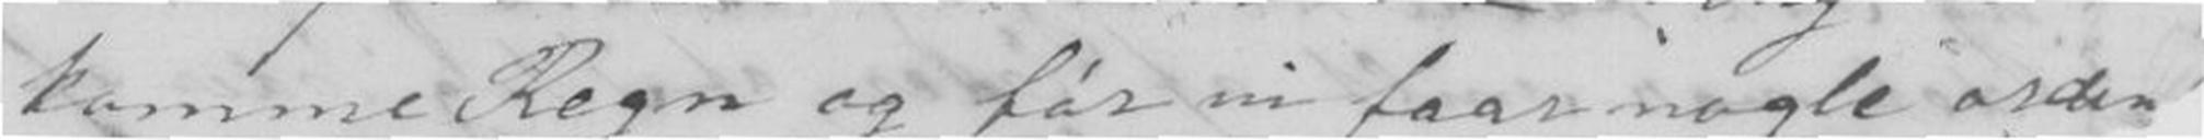komme Regn og før vi faar nogle orden
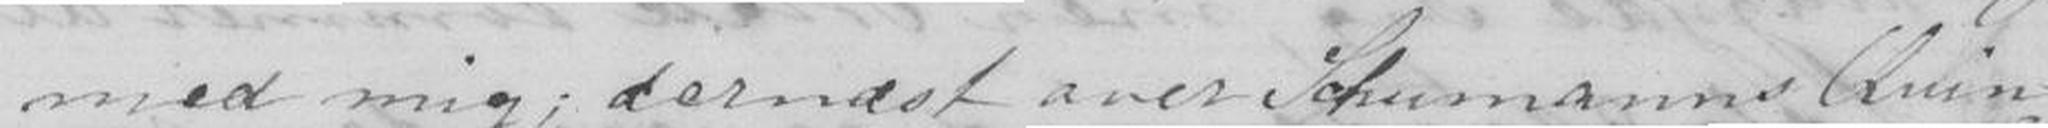med mig; dernæst over Schumsnns Qvin-
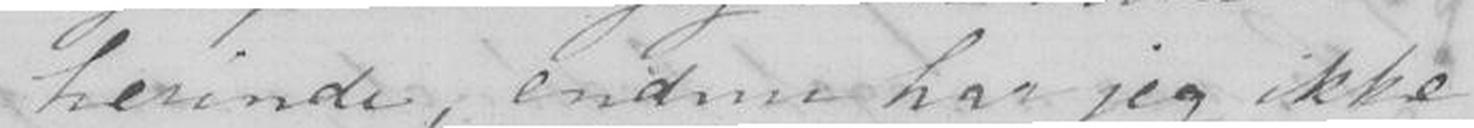herinde, endnu har jeg ikke
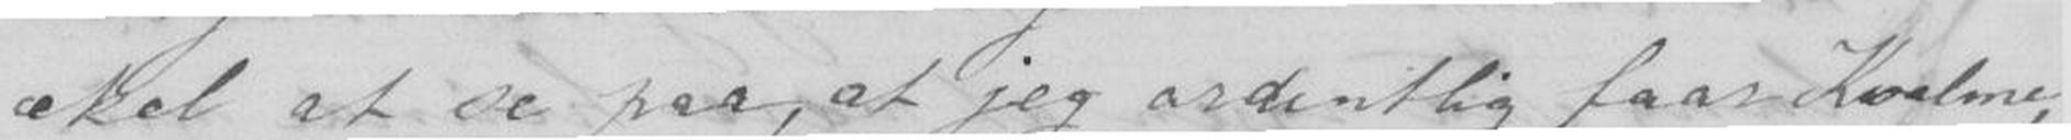ækel at se paa, at jeg ordentlig faar Kvalme,
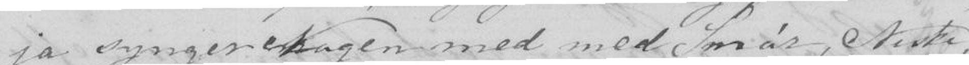ja synger Nogen med med Smør, Niske
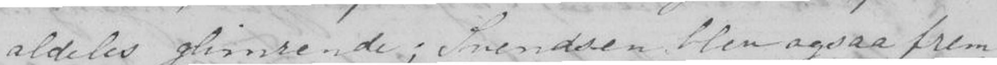aldeles glimrende; Svendsen blev ogsaa frem-
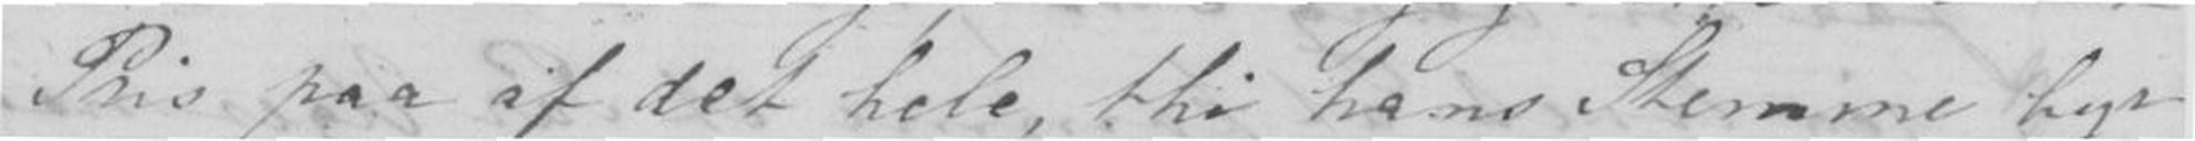Pris paa af det hele, thi hans Stemme byr
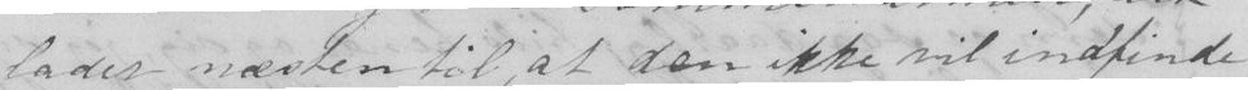mærket noget til, at den ikke vil indfinde
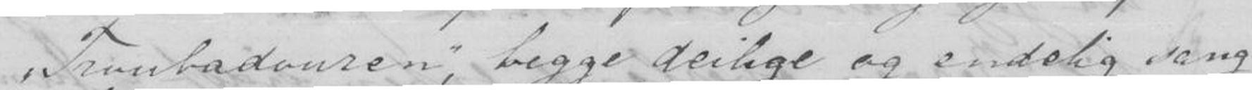Troubadouren, begge deilige og endelig sang
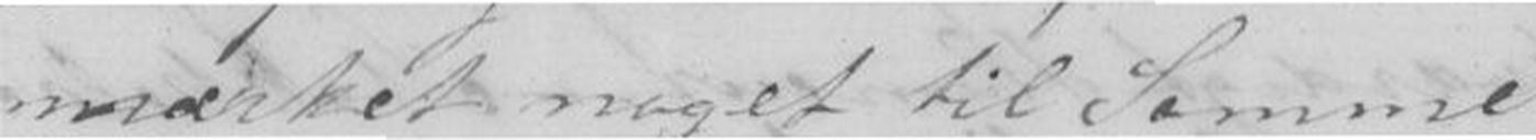mærket noget til Samme
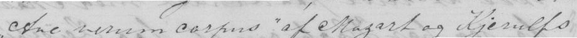Aue verum corpus af Mozart og Kjerulfs
